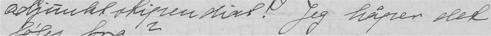adjunktstipendiat! Jeg håper det
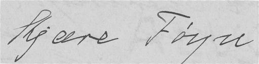Kjære Føyn
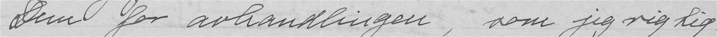Dem for avhandlingen, som jeg rigtig
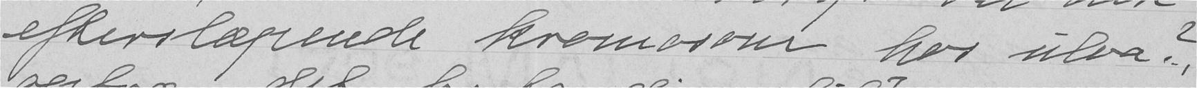efterslæpende kromosom hos ulva?,
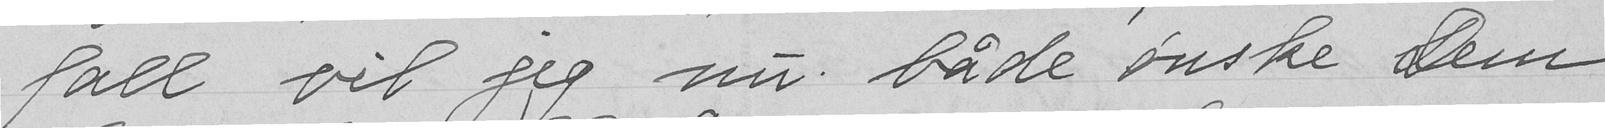fall vil jeg nu både ønske Dem
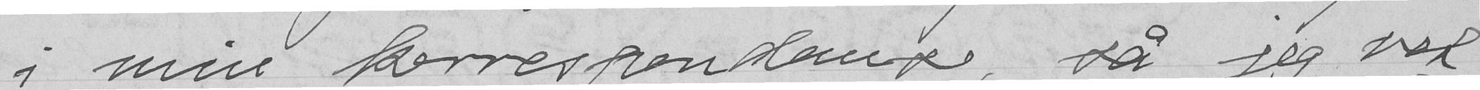i min korrespondanse, så jeg vet
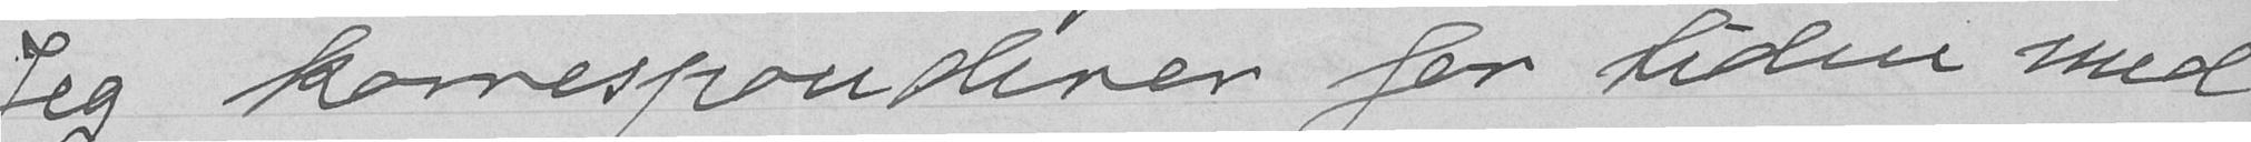Jeg korresponderer for tiden med
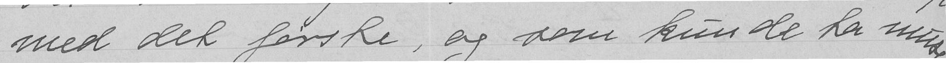med det første, og som kunde ta musene
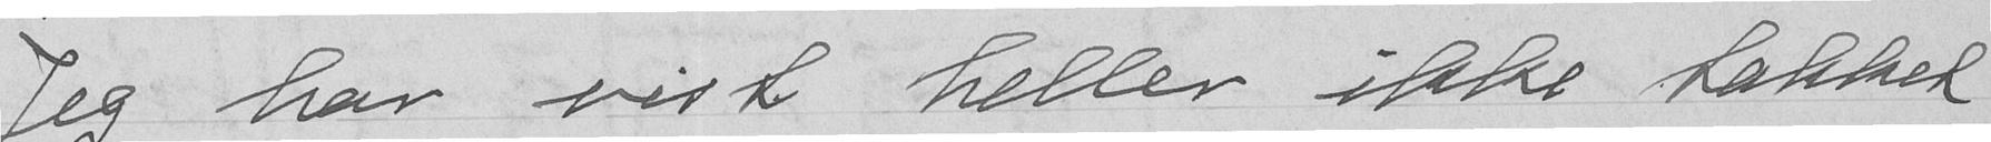Jeg har vist heller ikke takket
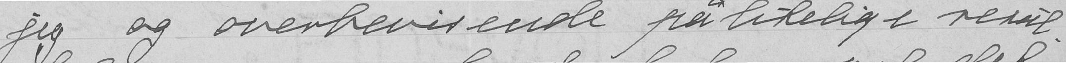jeg og overbevisende pålitelige resul-
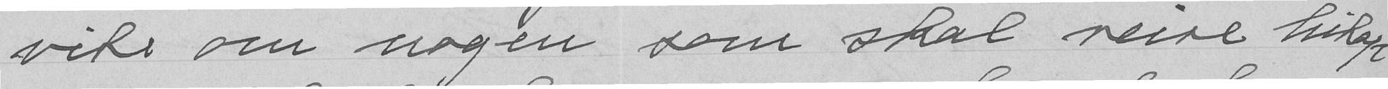vite om nogen som skal reise hitop
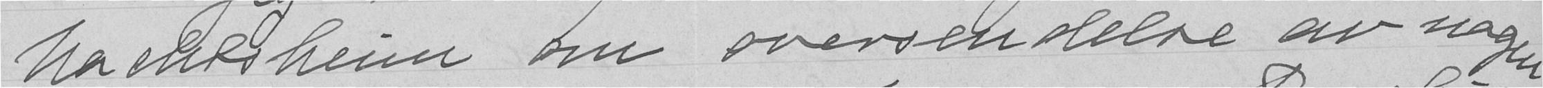Nachtsheim om oversendelse av nogen
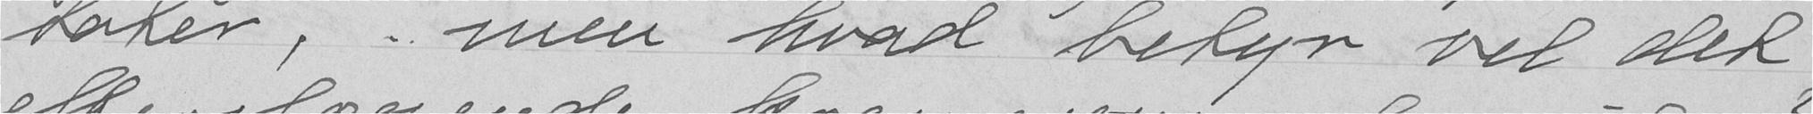tater,  men hvad betyr vel det
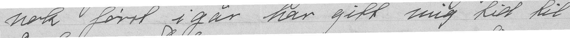nok først igår har gitt mig tid til
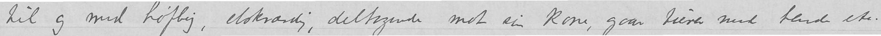til og med høflig, elskværdig, deltagende mot sin kone, gaar turer med hende etc.
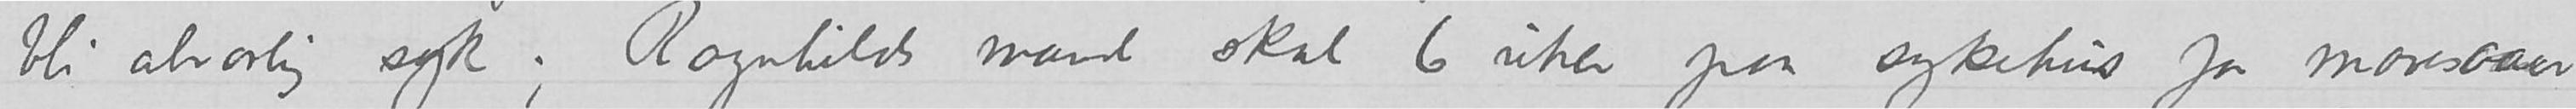bli alvorlig syk; Ragnhilds mand skal 6 uker paa sykehus for mavesaaer
